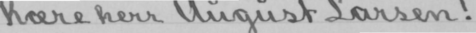Kære herr August Larsen!
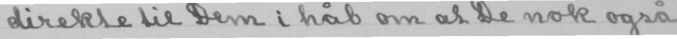direkte til Dem i håb om at De nok også
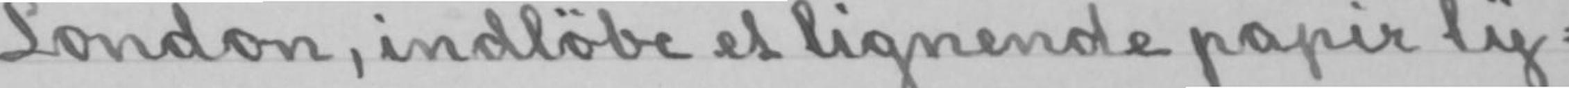London, indløbe et lignende papir ly-
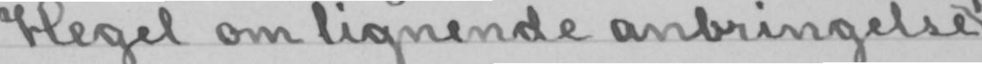Hegel om lignende anbringelse
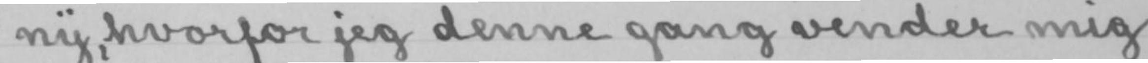ny, hvorfor jeg denne gang vender mig
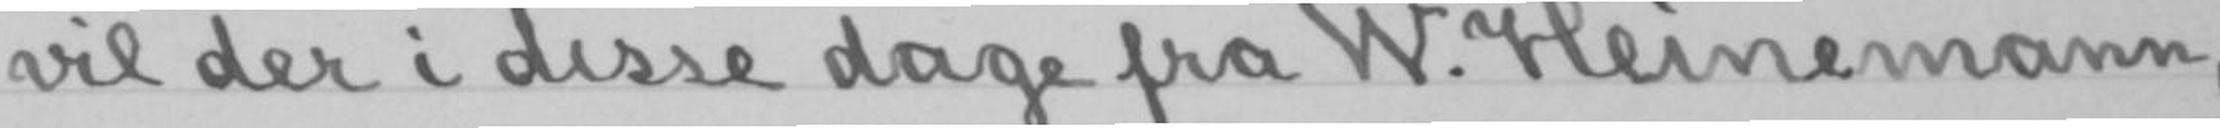vil der i disse dage fra W. Heinemann,
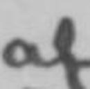af
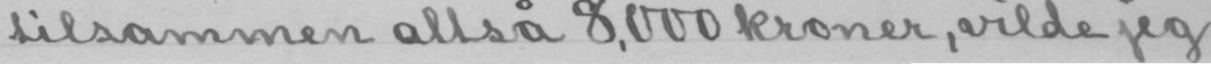tilsammen altså 8,000 kroner, vilde jeg
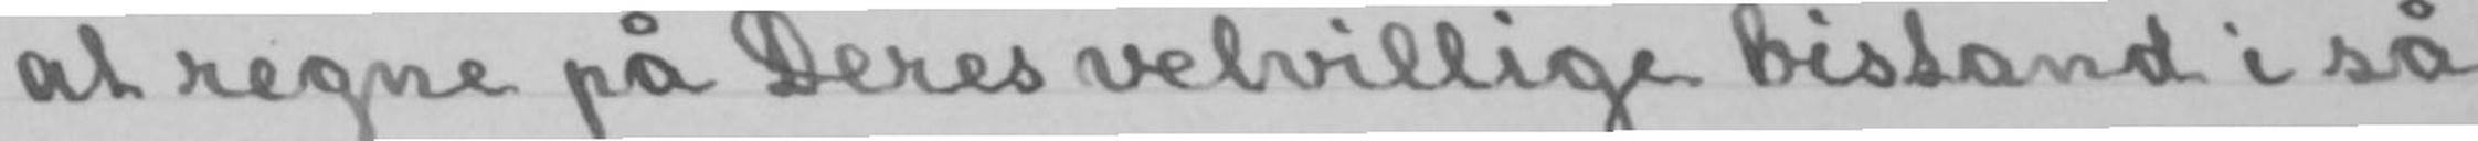at regne på Deres velvillige bistand i så
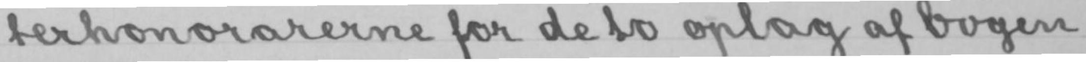terhonorarerne for de to oplag af bogen
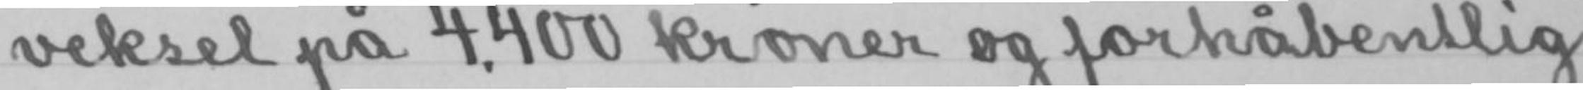veksel på 4,400 kroner og forhåbentlig
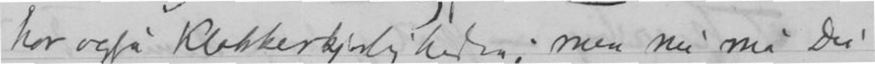har også Klokkekjerligheden; men nu må Du
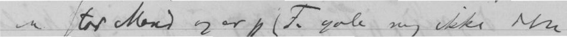en stor Mand og er jeg (F. gale mig ikke denne
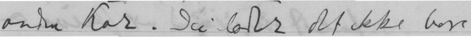anden Kar. Du lader det ikke bare
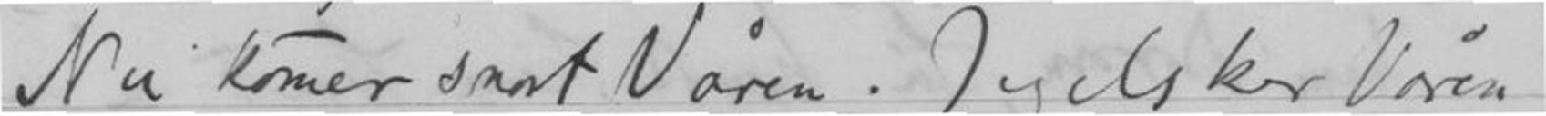Nu komer snart Våren. Jeg elsker Våren
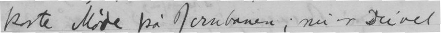korte Møde på Jernbanen; nu er Du vel
